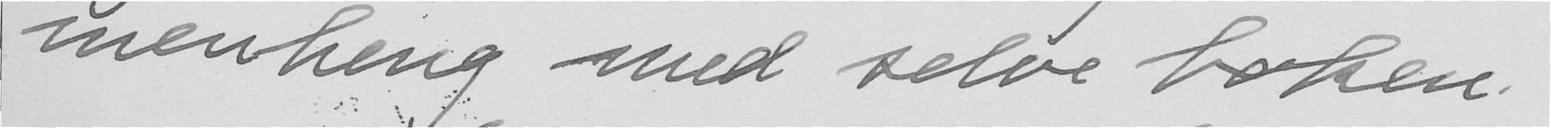menheng med selve boken.
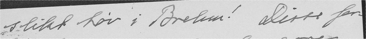slikt tøv i Berlin. Disse for-
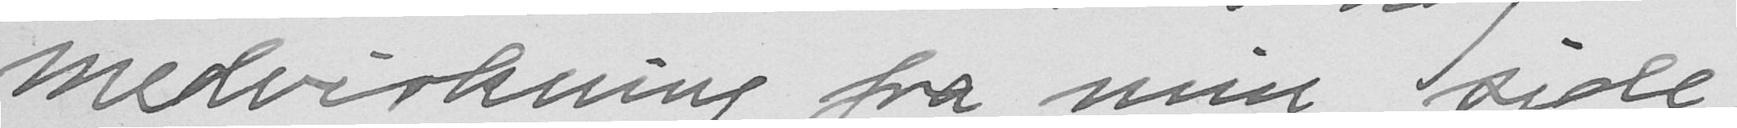medvirkning fra min side
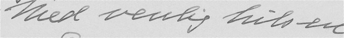Med venlig hilsen
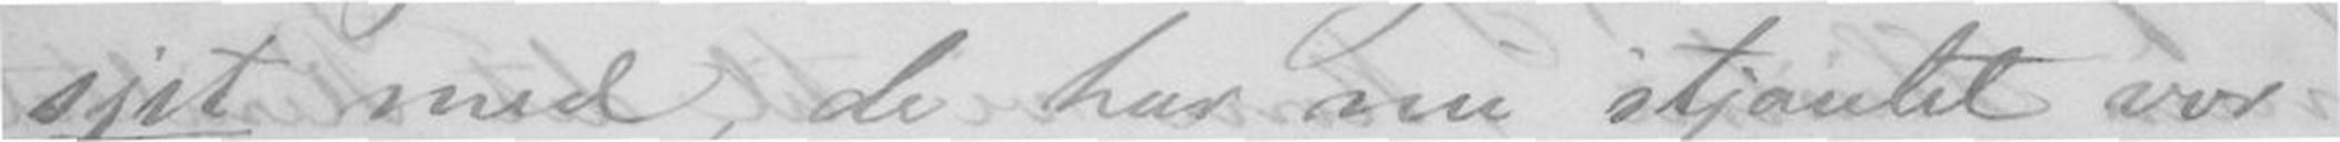sjet med, de har nu stjanlet vor
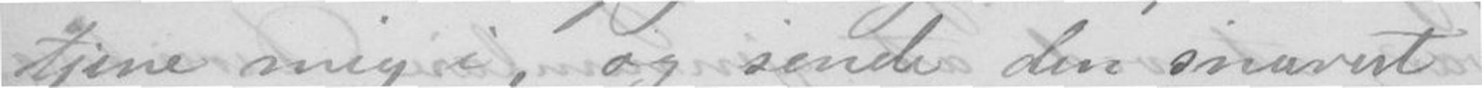tjene mig i, og sende den snarest
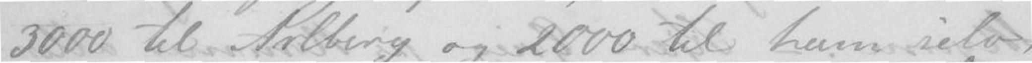3000 til Arlberg og 2000 til ham selv,
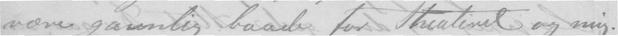være gavnlig baade for Theateret og mig.
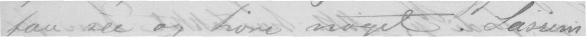faa see og høre noget. Lassens
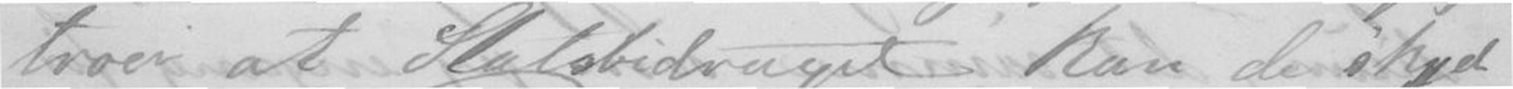tror at Statsbidraget kan de skyd
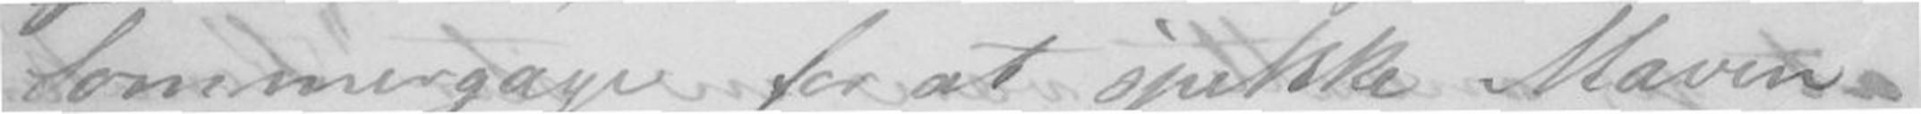Sommergage for at spekke Maven
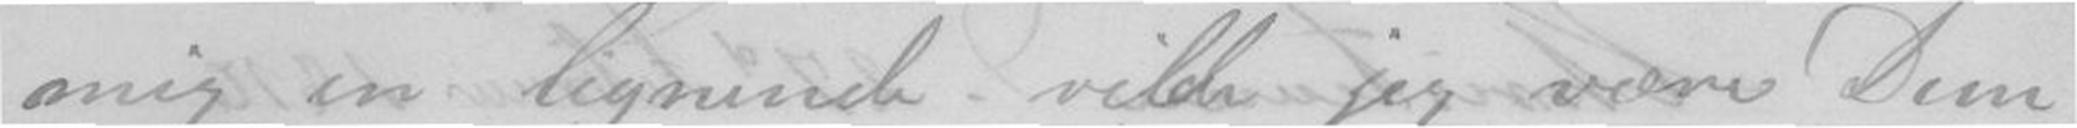mig en lignende vilde jeg være Dem
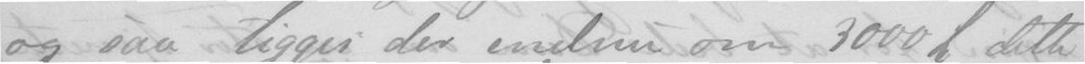og saa ligger der endnu om 3000 £. dette
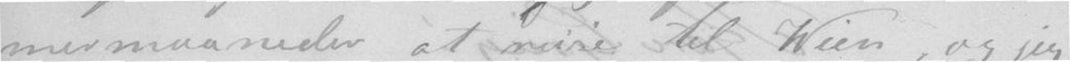mermaaneder at reise til Wien, og jeg
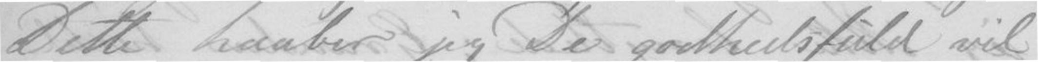Dette haaber jeg De godhedsfuld vil
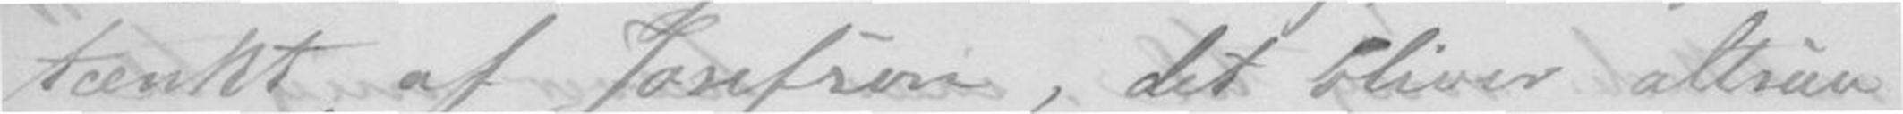tænkt af Josefson, det bliver altsaa
